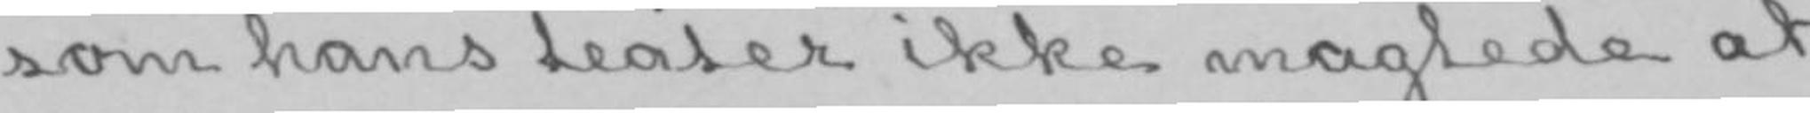som hans teater ikke magtede at
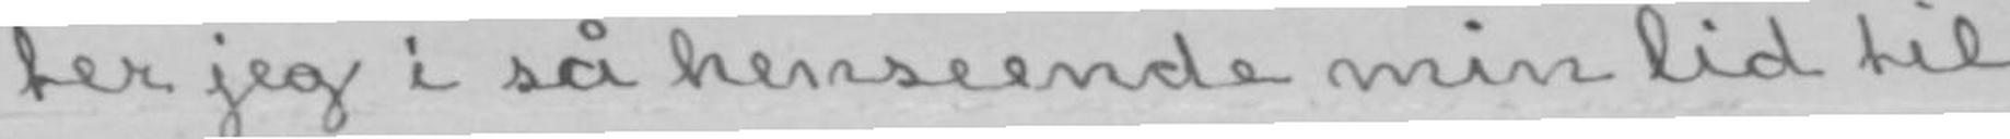ter jeg i så henseende min lid til
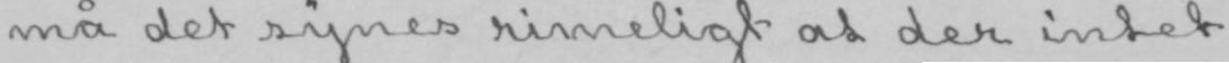må det synes rimeligt at der intet
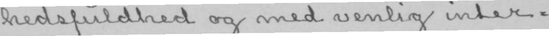hedsfuldhed og med venlig inter-
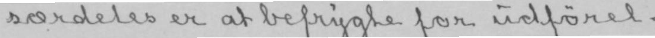særdeles er at befrygte for udførel-
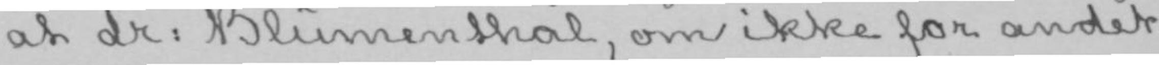at dr: Blumenthal, om ikke for andet
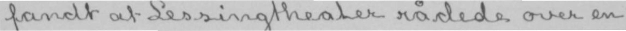fandt at Lessingtheater rådede over en
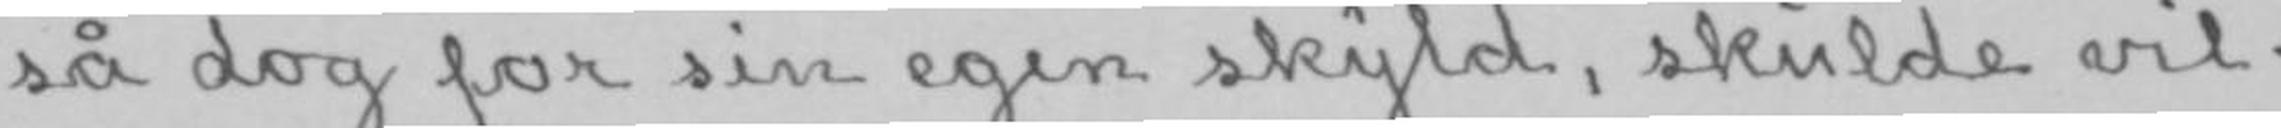så dog for sin egen skyld, skulde vil-
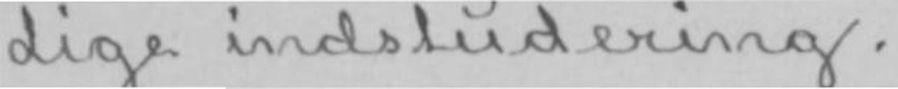dige indstudering.
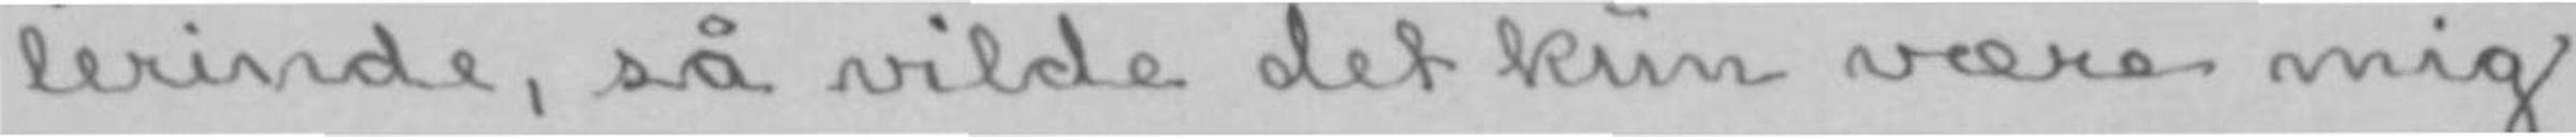lerinde, så vilde det kun være mig
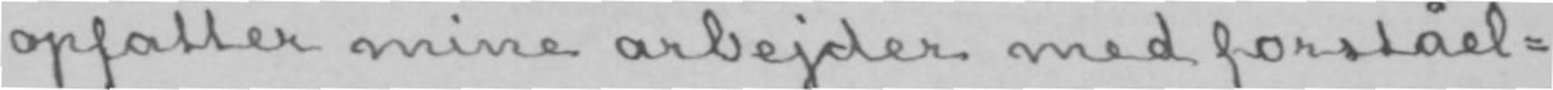opfatter mine arbejder med forståel-
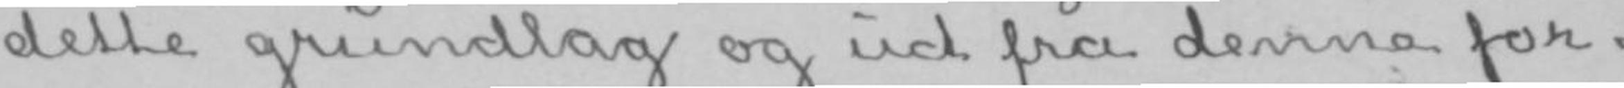dette grundlag og ud fra denne for-
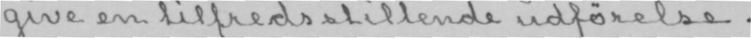give en tilfredsstillende udførelse.
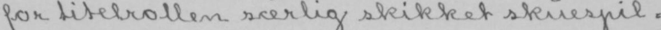for titelrollen særlig skikket skuespil-
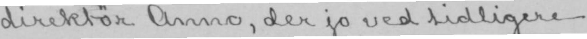direktør Anno, der jo ved tidligere
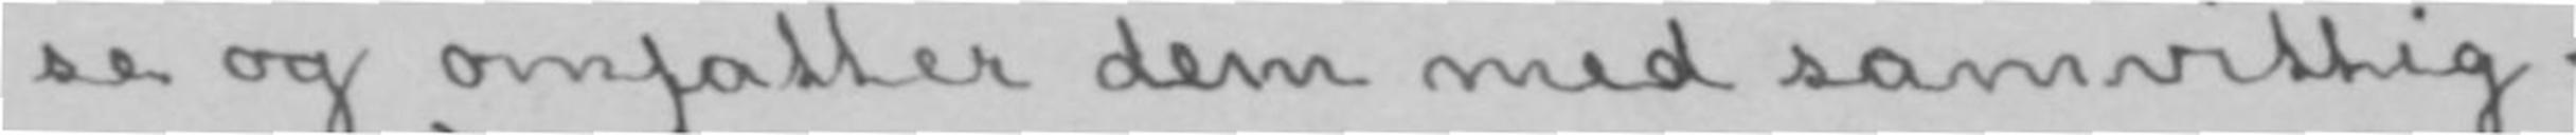se og omfatter dem med samvittig-
